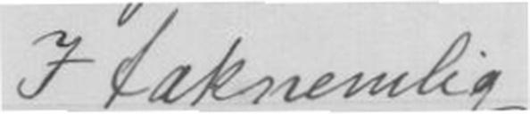I taknemlig
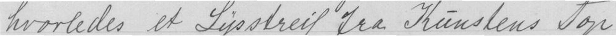hvorledes et Lysstreif fra Kunstens Top
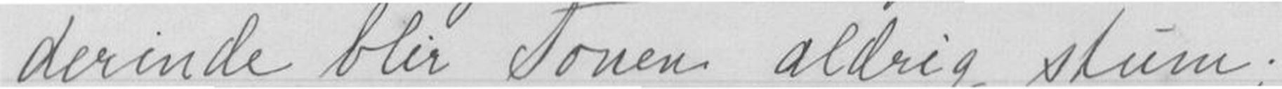derinde blir Tonen aldrig stum;
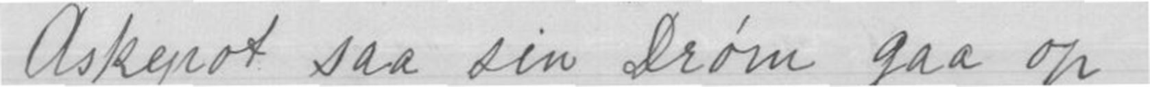Askepot saa sin Drøm gaa op -
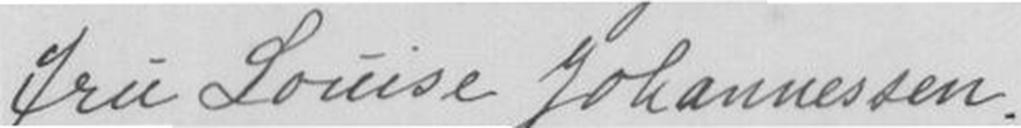Fru Louise Johannessen
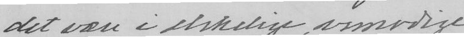det være i elskelige, vemodige
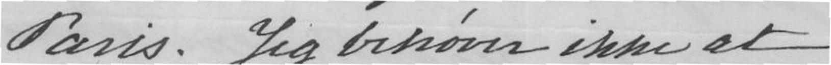Paris. Jeg behøver ikke at
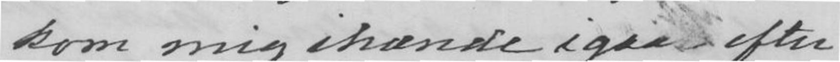kom mig ihænde igaar efter
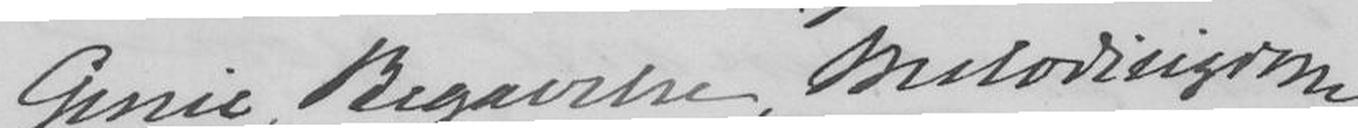Genie, Begavelse, Melodirigdom
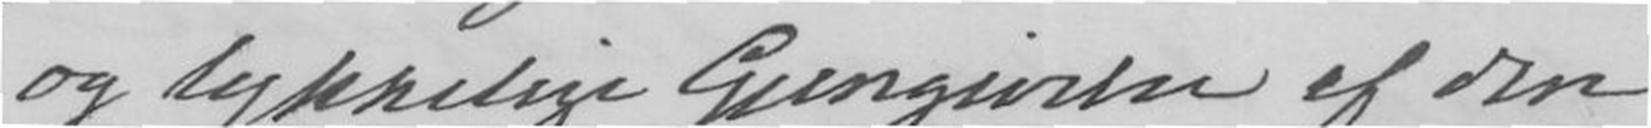og lykkelige Gjengivelse af den
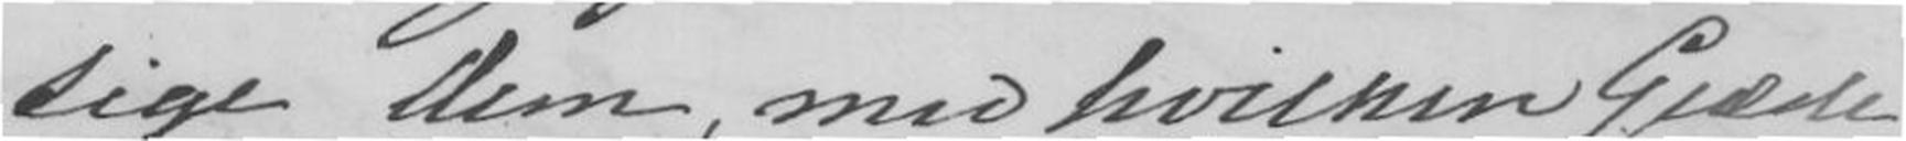sige Dem, med hvilken Glæde
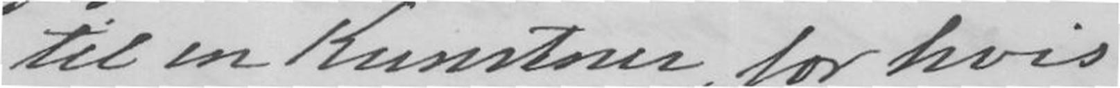til en Kunstner, for hvis
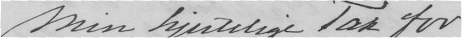Min hjertelige Tak for
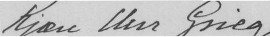Kjære Herr Grieg
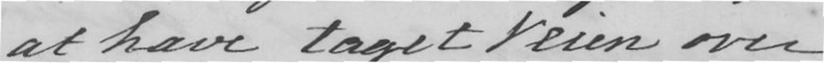at have taget Veien over
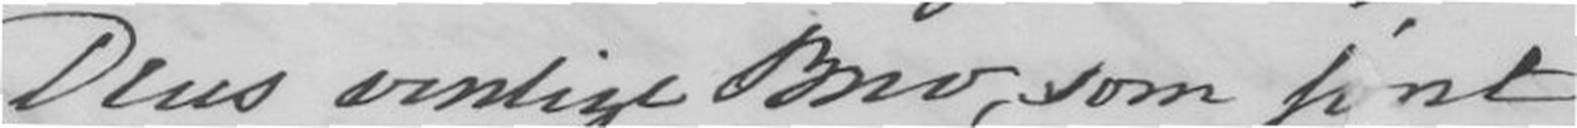Deres venlige Brev, som først
